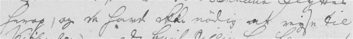herop, og de havde ikke nødig at reyse til
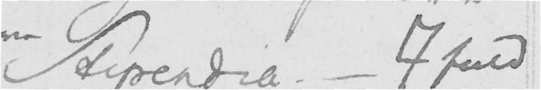Stipendia. I fald
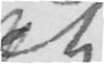et
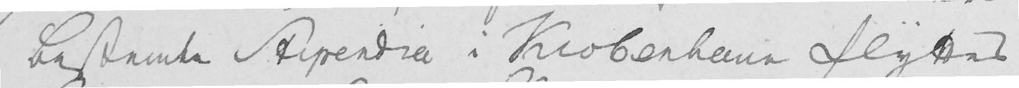bestemte Stipendia i Kiøbenhaun flyttes
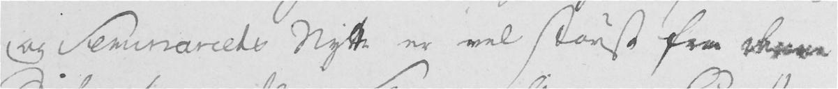og Seminariets Nytte er vel størst fra deres
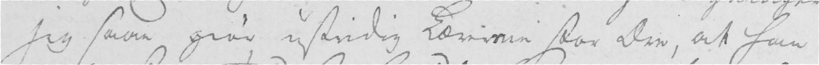jeg saae giør ustridig Læreren stor Dre, at han
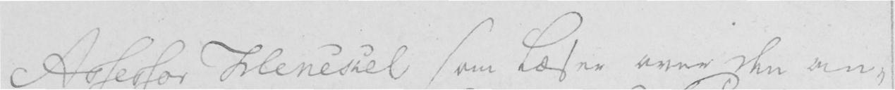Assessor Henckel som læser over den an-
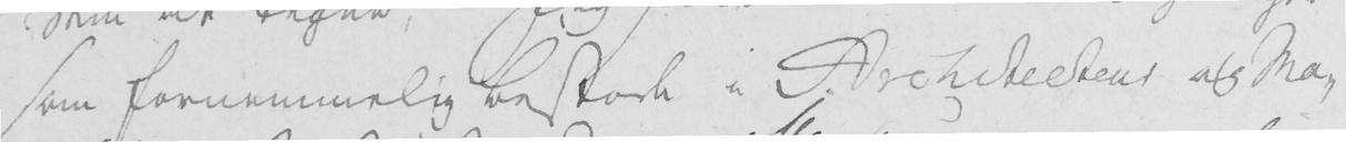som fornemmelig bestode i Architecteur og Ma-
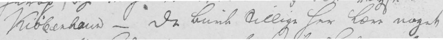Kiøbenhaun - De burde tillige her lære noget
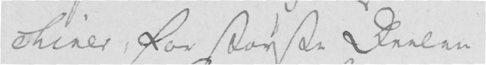chiner, for største Deelen
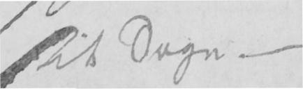sit Sogn. -
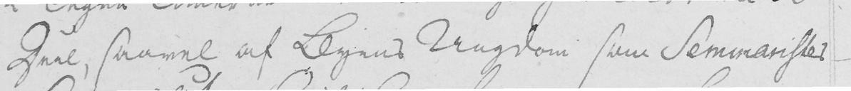Deel, saavel af Byens Ungdom som Seminarister -
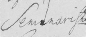Seminarist
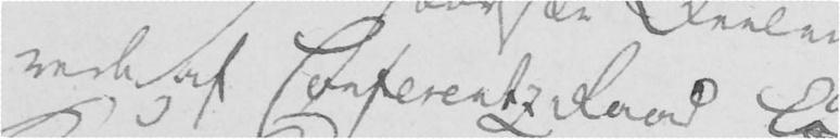rede af Conferentz Raad C
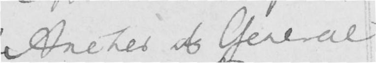Ancher og Gereal
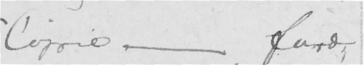Copie - ford-
